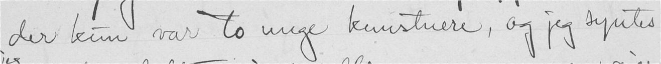der kun var to unge kunstnere, og jeg syntes
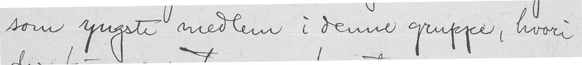som yngste medlem i denne gruppe, hvori
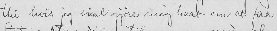thi hvis jeg skal gjøre mig haab om at faa
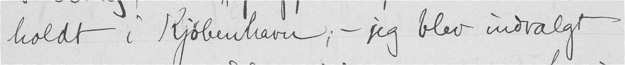holdt i Kjøbenhavn; - jeg blev indvalgt
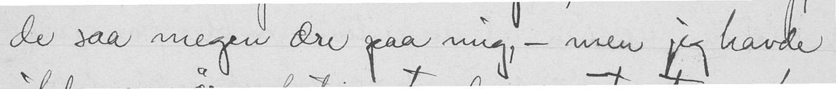de saa megen ære paa mig, - men jeg havde
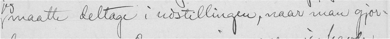maatte deltage i udstillingen, naar man gjor-
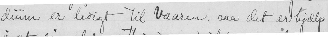dium er ledigt til vaaren, saa det er hjælp
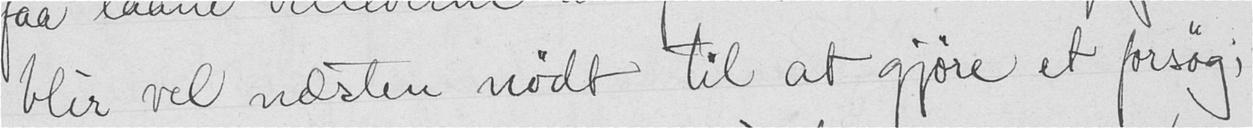blir vel næsten nødt til at gjøre et forsøg;
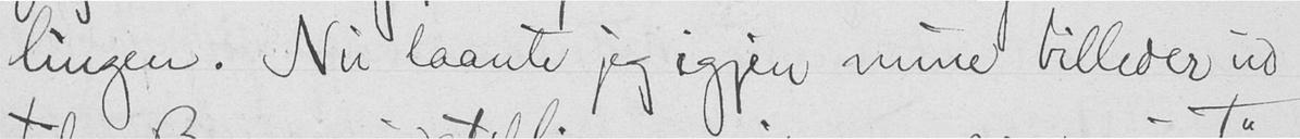lingen. Nu laante jeg igjen mine billeder ud
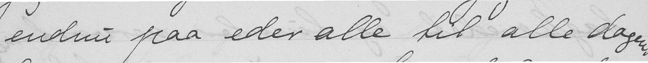endnu paa eder alle til alle dagens
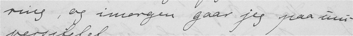ring, og imorgen gaar jeg paa uni-
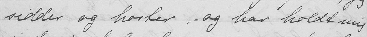sidder og hoster, - og har holdt mig
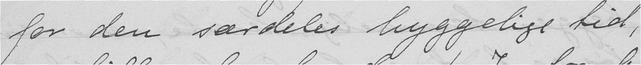for den særdeles hyggelige tid,
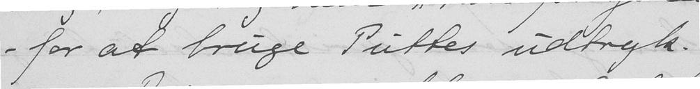- for at bruge Puttes udtryk.
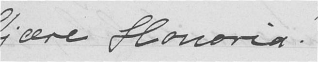Kjære Honoria!
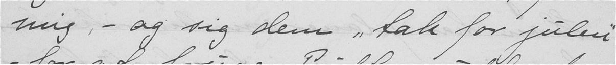mig, - og sig dem,"tak for julen"
